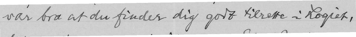var bra at du finder dig godt tilrette i Logiet,
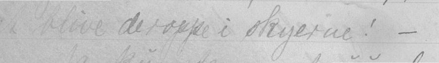at blive deroppe i skyerne!
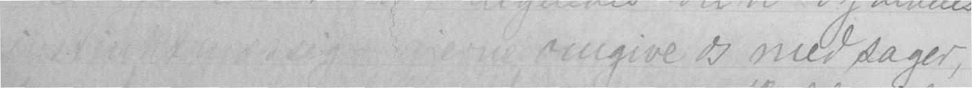instinktmæssig gjerne omgive os med sager,
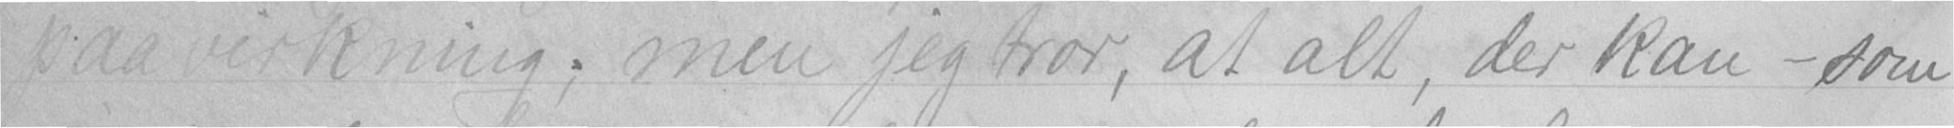paavirkning; men jeg tror, at alt, der kan - som
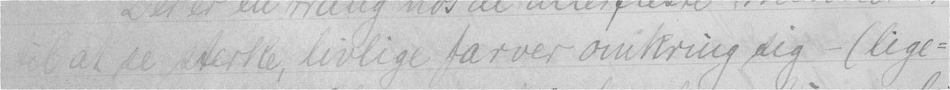til at se sterke, livlige farver omkring sig - (lige-
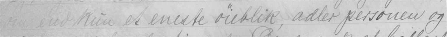om end kun et eneste øieblik, adler personen og
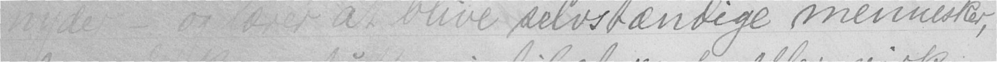nyder - og lærer at blive selvstændige mennesker,
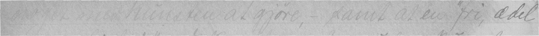noget med kunsten at gjøre, - samt at en "fri, ædel
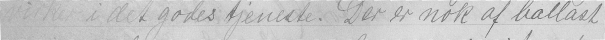virker i det godes tjeneste. Der er nok af ballast
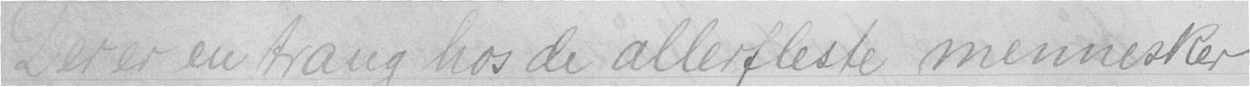Der er en trang hos de allerfleste mennesker
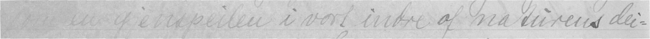som en gjenspeilen i vort indre af naturens dei-
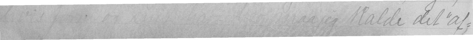hvis form og  her maa jeg kalde det "af
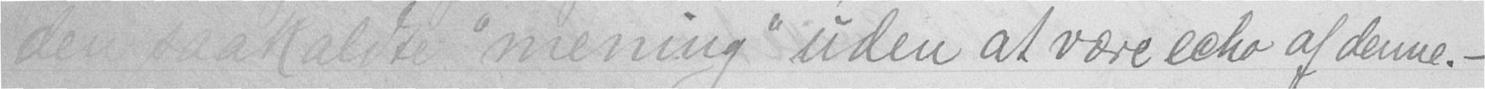den saakaldte "mening" uden at være echo af denne. -
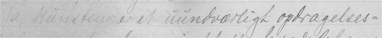Ja, kunsten er et uundværligt opdragelses-
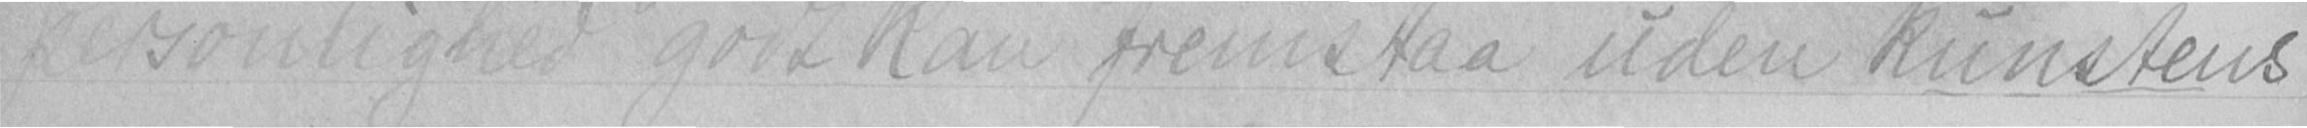personlighed" godt kan fremstaa uden kunstens
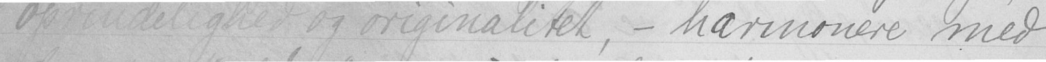oprindelighed og originalitet, - harmonere med
